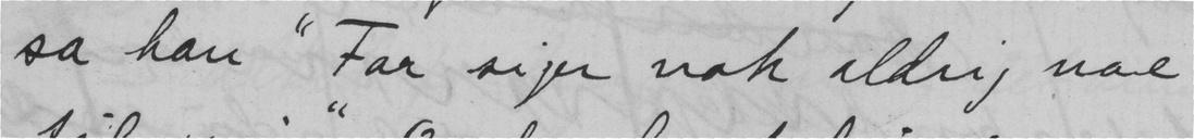sa han "Far siger nok aldrig noe
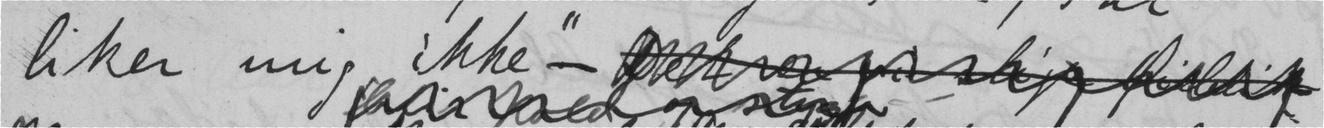liker mig ikke" -
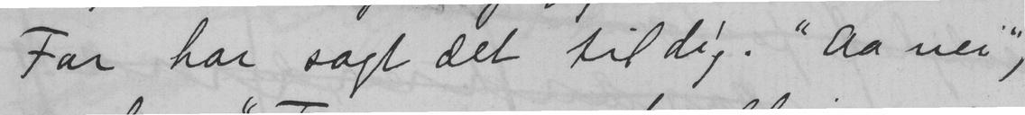Far har sagt det til dig. "Aa nei",
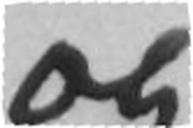og
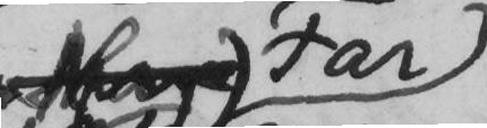Far
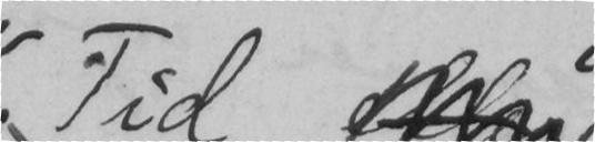Tid
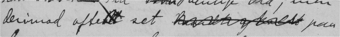derimod ofte set  paa
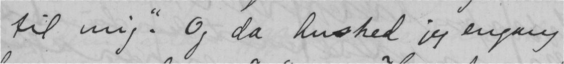til mig". Og da husked jeg engang
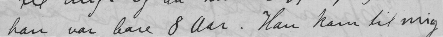han var bare 8 aar. Han kom til mig
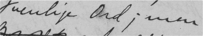venlige Ord; men
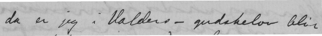da er jeg i Valders - gudskelov blir
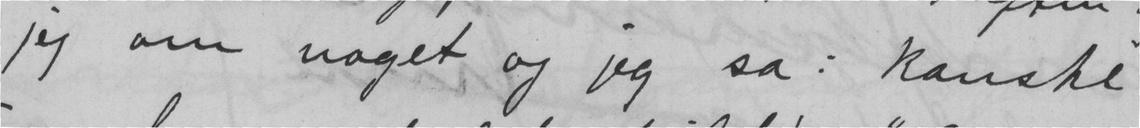jeg om noget og jeg sa: kanske
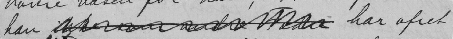han  har ofret
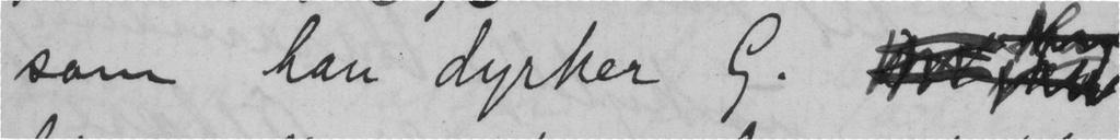som han dyrker G.
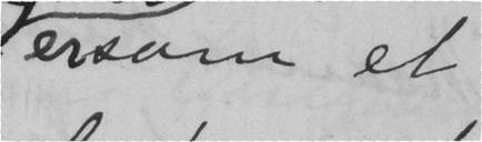er som et
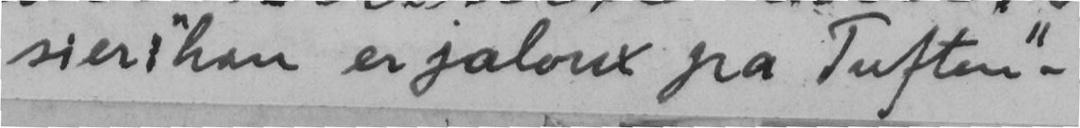sier "han er jaloux på Tuften" -
